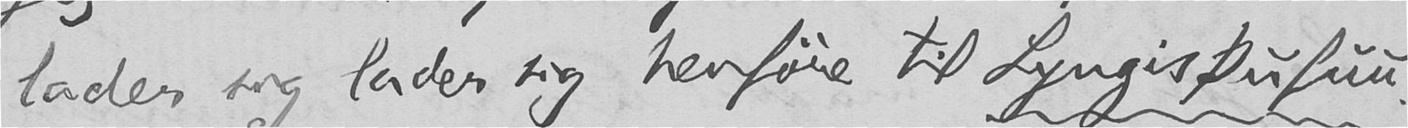lader sig lader sig henføre til Lynghesþufuu.
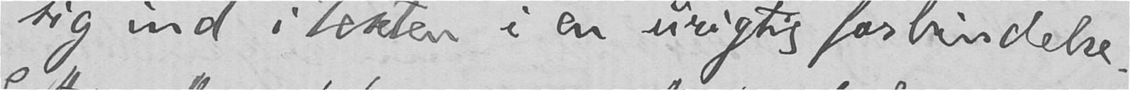sig ind i texten i en urigtig forbindelse.
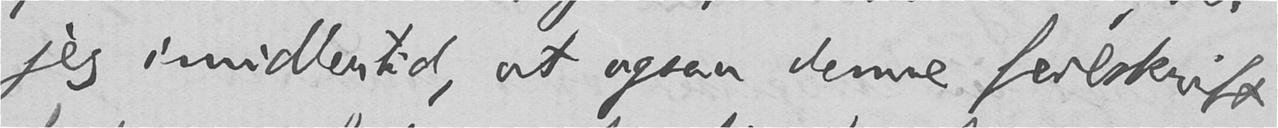jeg imidlertid, at ogsaa denne feilskrift
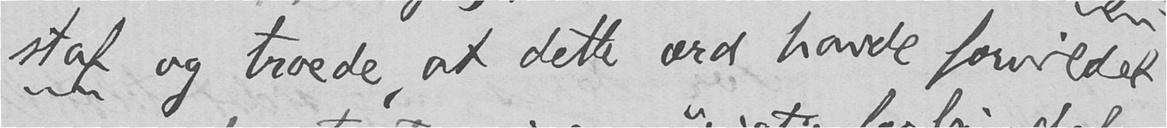staf og troede, at dette ord havde forvildet
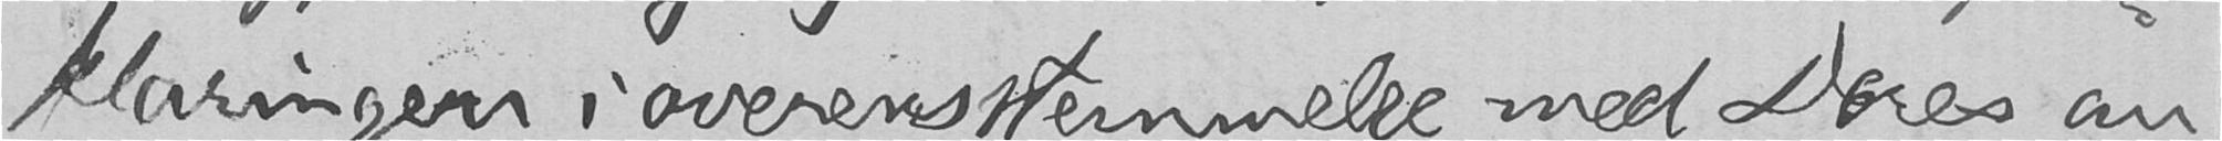klaringen i overensstemmelse med Deres an-
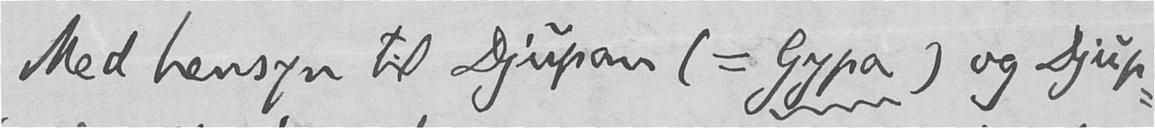Med hensyn til Djupan (= Gypa) og Djup-
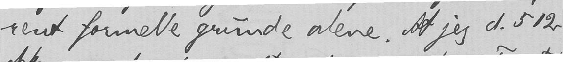rent formelle grunde alene. At jeg d. 512
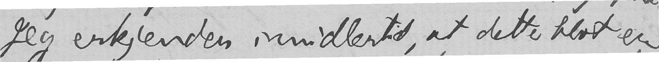Jeg erkjender imidlertid at dette blot er
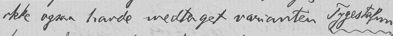ikke ogsaa havde medtaget varianten Tygestafnn
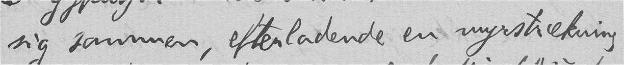sig sammen, efterladende en myrstrækning
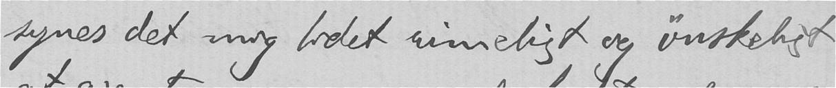synes det mig lidet rimeligt og ønskeligt
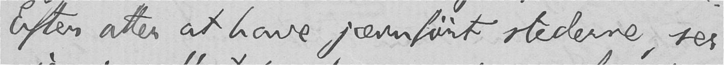Efter atter at have jævnført stederne, ser
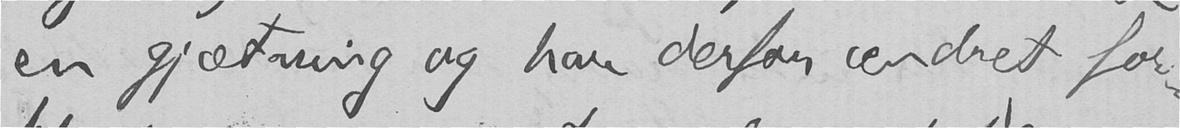en gjætning og har derfor ændret for-
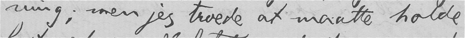ning; men jeg troede at maatte holde
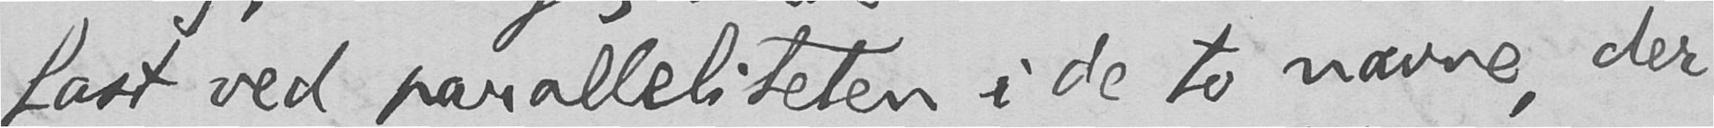fast ved paralleliteten i de to navne, der
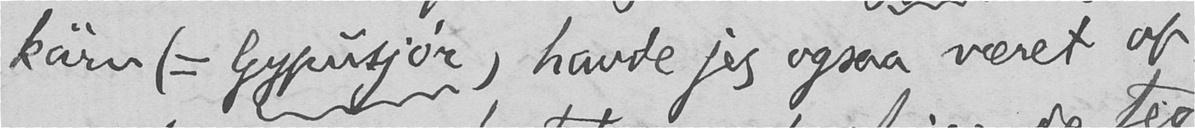kärn (= Gypusjór) havde jeg ogsaa været op-
